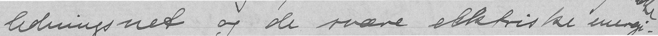ledningsnet og de svære elektriske energi-
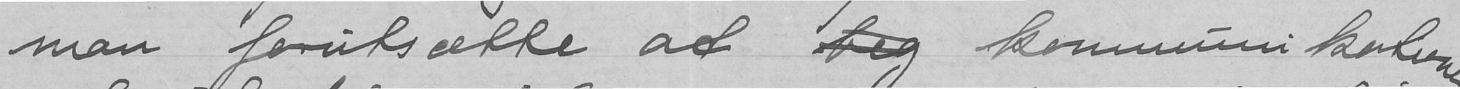man forutsætte at beg kommunikationene
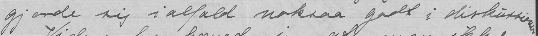gjorde sig ialfald noksaa godt i diskussionen
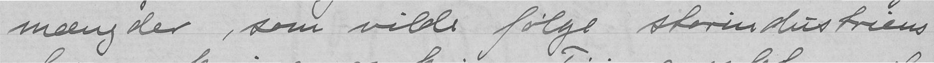mængder, som vilde følge storindustriens
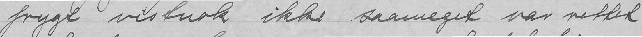frygt vistnok ikke saameget var rettet
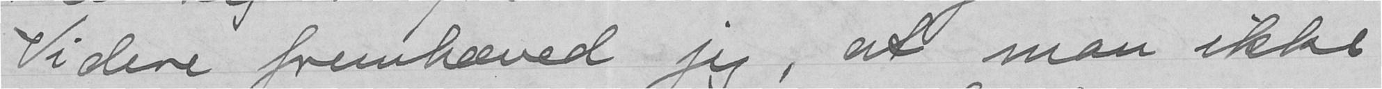Videre fremhæved jeg, at man ikke
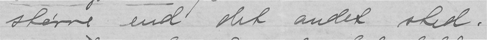større end det andet sted.
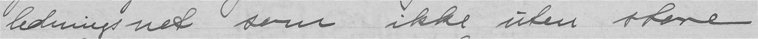ledningsnet som ikke uten store
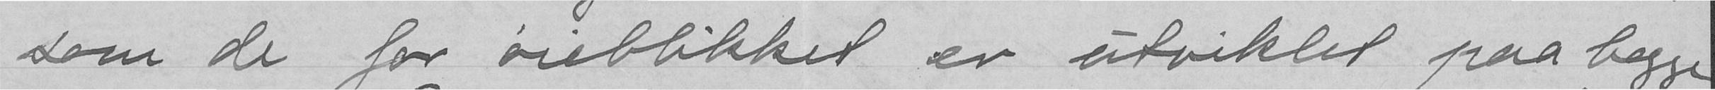som de for øieblikket er utviklet paa begge
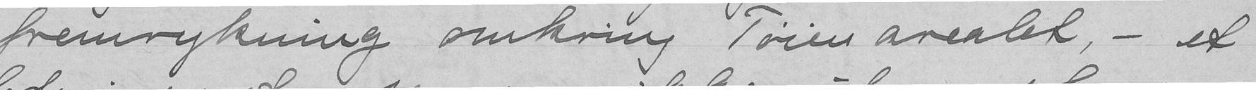fremrykning omkring Tøienarealet, - et
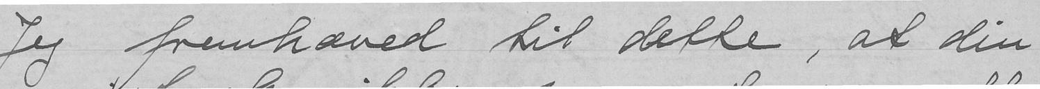Jeg fremhæved til dette, at din
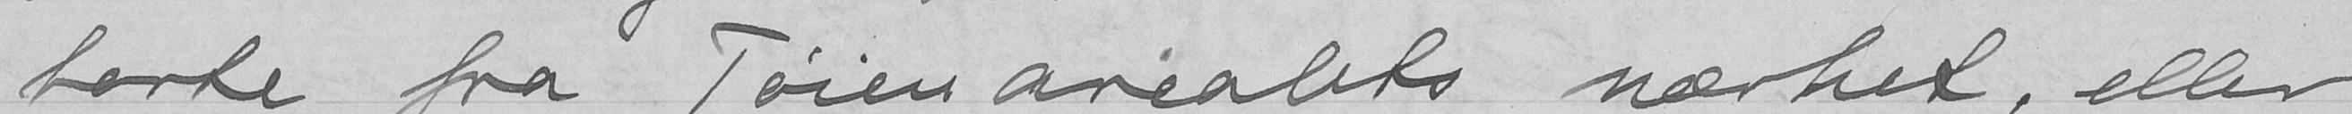borte fra Tøienarealets nærhet, eller
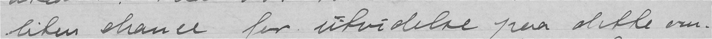liten chance for utvidelse paa dette om-
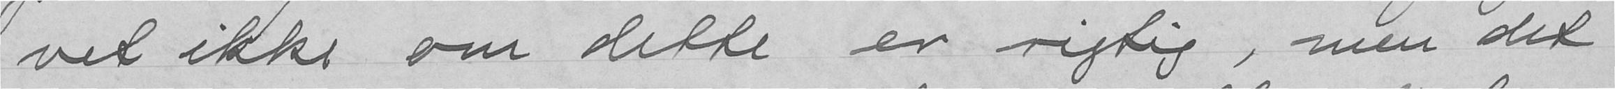vet ikke om dette er rigtig, men det
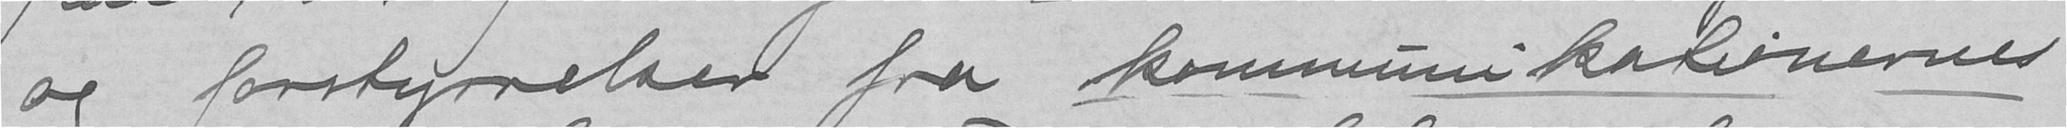og forstyrrelser fra kommunikationernes
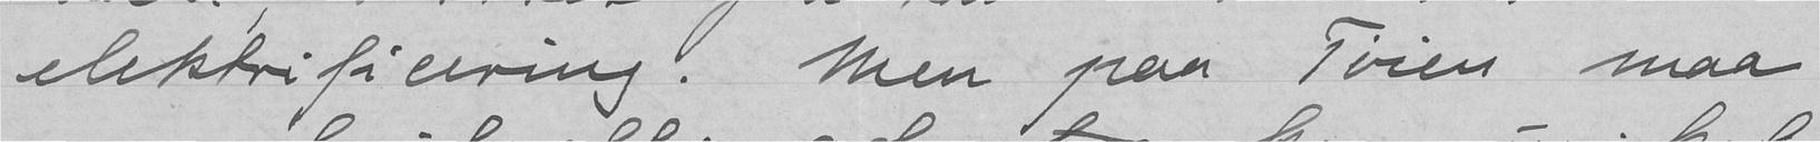elektrificering. Men paa Tøien maa
